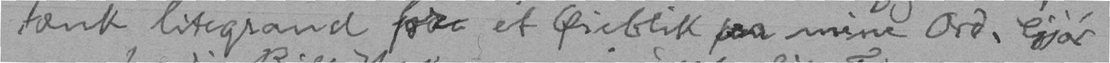tænk litegrand for et Øieblik paa mine Ord. Gjør
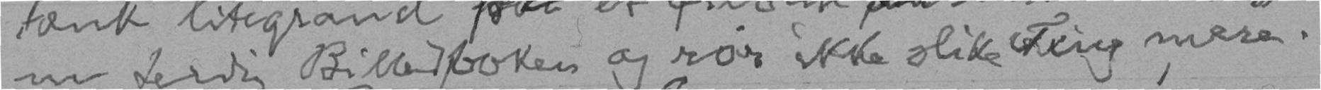nu ferdig Billedboken og rør ikke slike Ting mere.
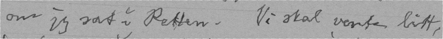om jeg sat i Retten. Vi skal vente litt,
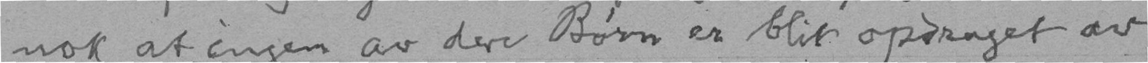nok at ingen av dere Børn er blit opdraget av
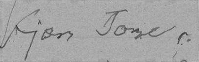Kjære Tore,.
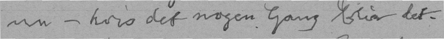nu - hvis det nogen Gang blir det.
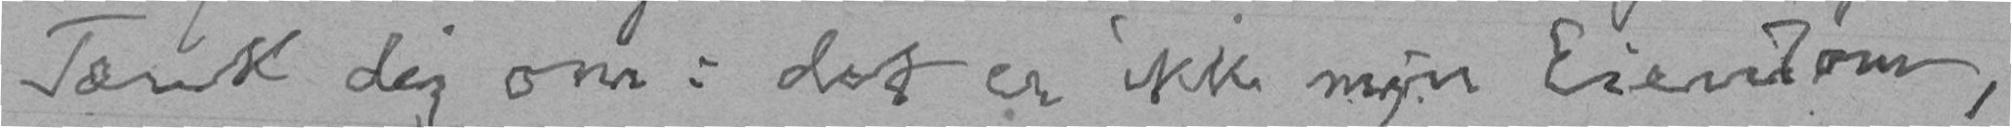Tænk dig om: det er ikke min Eiendom,
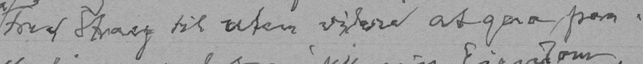Fru Stray til uten videre at gaa paa.
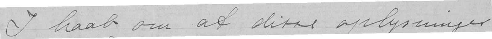I haab om at disse oplysninger
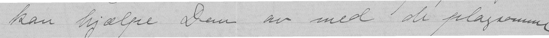kan hjælpe Dem av med de plagsomme
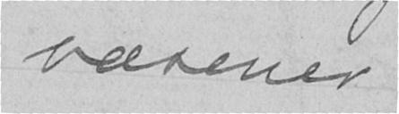væsener
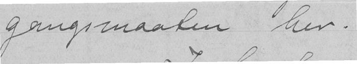gangsmaaten her.
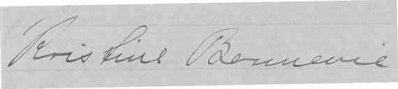Kristine Bonnevie
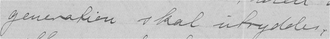generation skal udryddes.
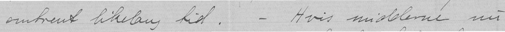omtrent likelang tid. - Hvis midderne nu
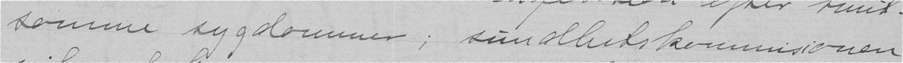somme sygdommer; sundhetskommisionen
